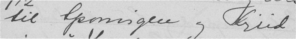til Sponvigen og Sigrid
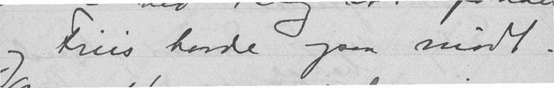og Friis havde ogsaa mødt.
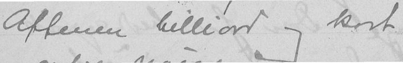Aftenen billiard og kort
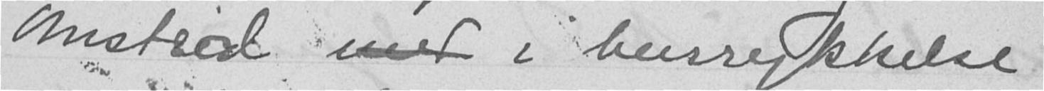Amstrid med i henrykkelse
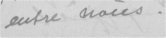entre hans.
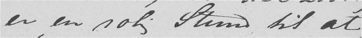er en rolig Stund til at
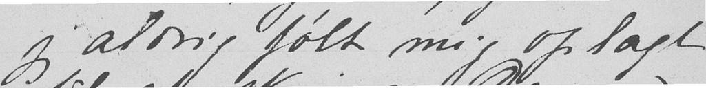jeg aldrig følt mig oplagt
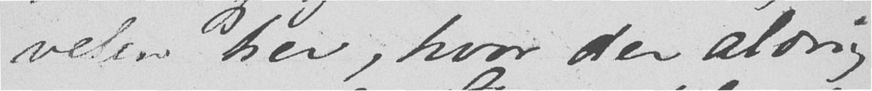velen her, hvor der aldrig
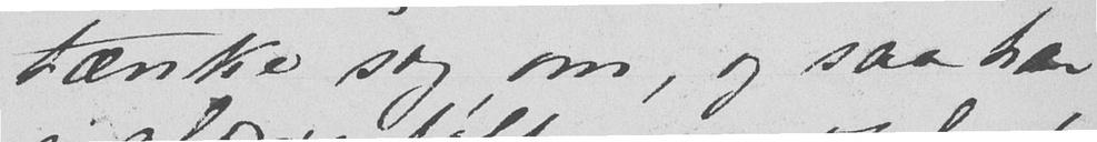tænke sig om, og saa har
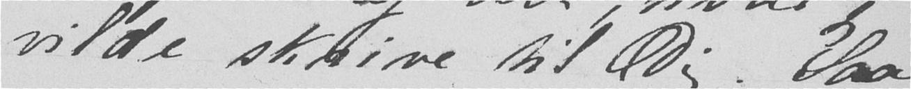vilde skrive til Dig. Saa
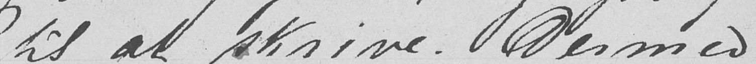til at skrive. Dermed
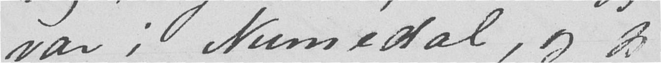var i Numedal, og jeg
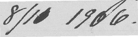8/10 1906.
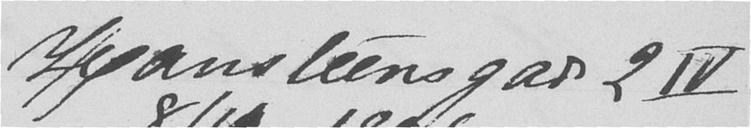Hansteensgade 2 IV
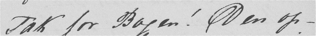Tak for Bogen! Den op-
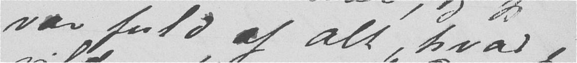var fuld af alt hvad jeg
